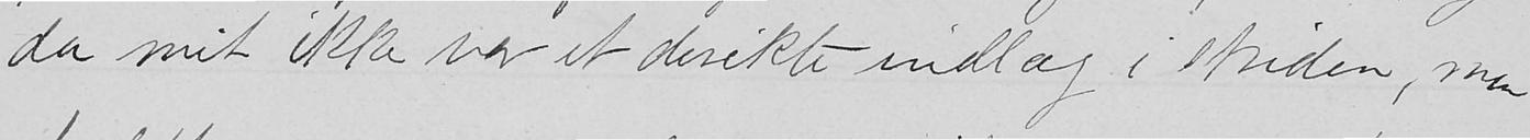da mit ikke var et direkte indlæg i striden, men
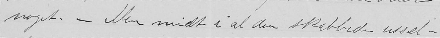noget. - Men midt i al den skabbede ussel-
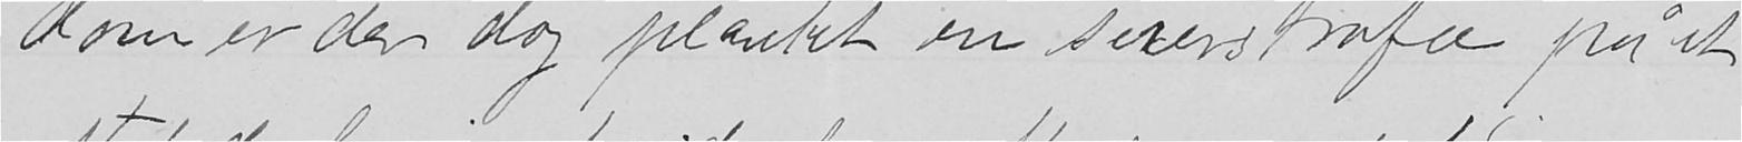dom er der dog plantet en seierstrofæ på et
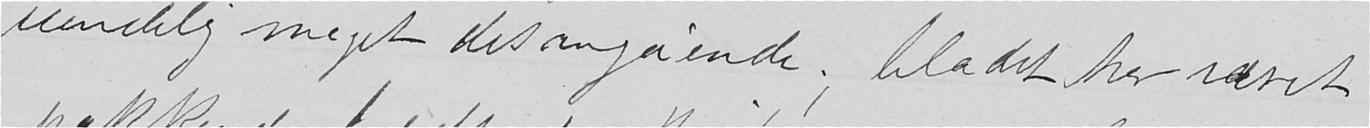uendelig meget desangående, bladet har været
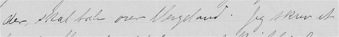der, skal tale over Vergeland. Jeg skrev et
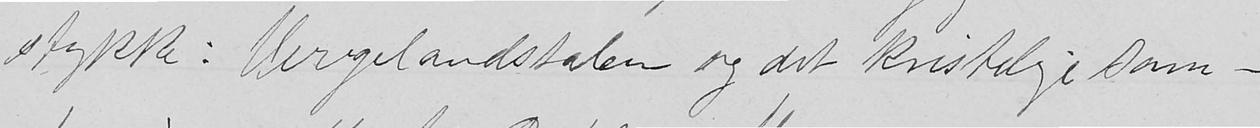stykke: Vergelandstalen og det kristelige sam-
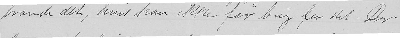brænde det, hvis han ikke får brug for det. Der
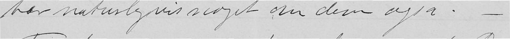var naturligvis noget om dem også. -
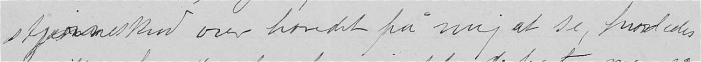stjerneskind over hovedet på mig at se, hvorledes
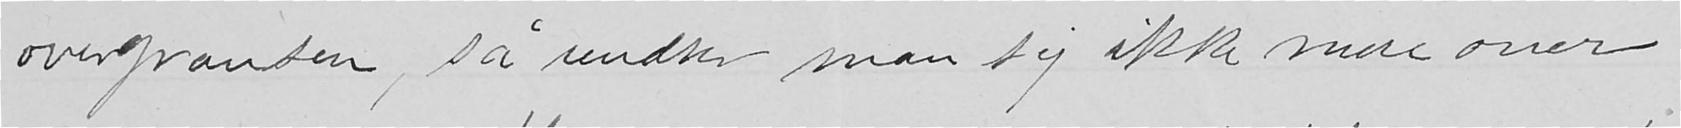overgrænsen, så undrer man sig ikke mere over
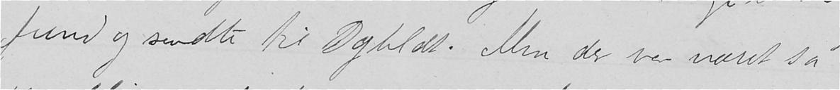fund og sendte til Dgbldt. Men der har været så
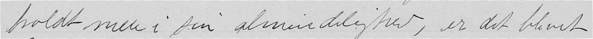holdt mere i sin almindelighed, er det blivet
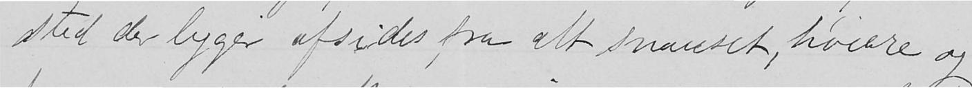sted der ligger afsides fra alt snauset, høiere og
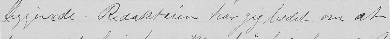liggende. Redaktøren har jeg bedet om at
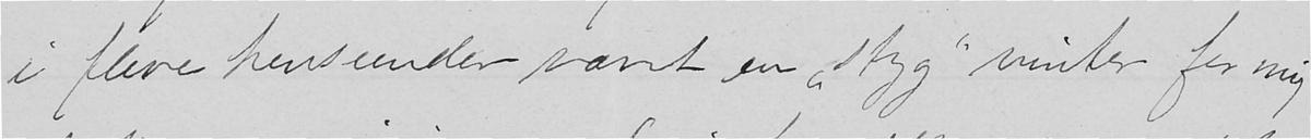i flere henseender været en "styg" vinter for mig
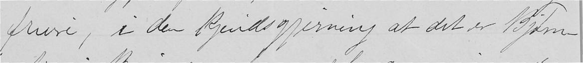friere, i den kjendsgjerning at det er Bjørn-
